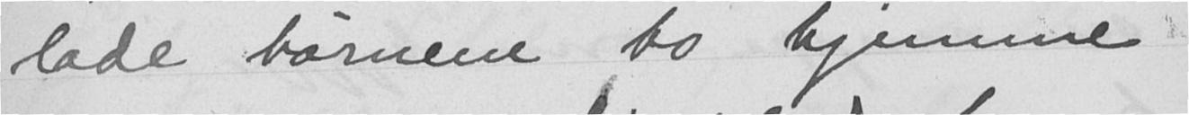lade børnene bo hjemme
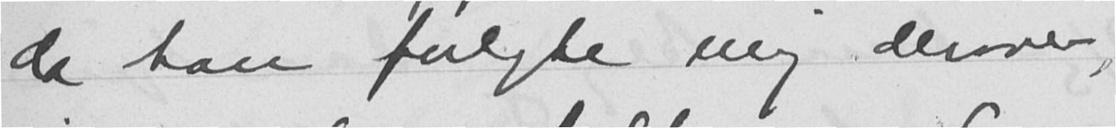da han fulgte mig derover,
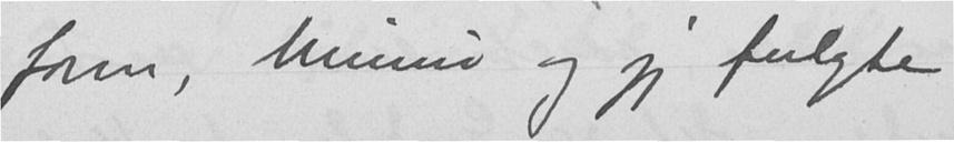form, Minni og jeg fulgte
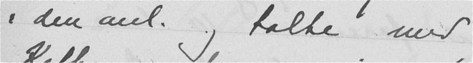i den anl. og talte med
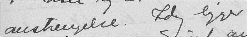anstrengelse. Idag ligger
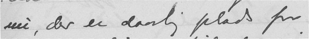nu, der er daarlig plads for
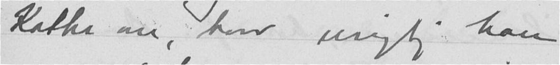Kathr om, hvor urigtig han
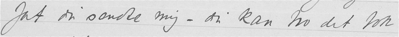fat du sendte mig - du kan tro det tok
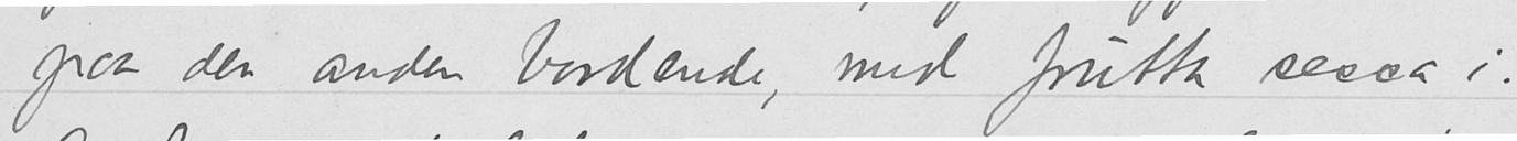paa den anden bordende, med frutta secca i!
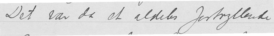Det var da et aldeles fortryllende
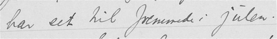har set til fremmede i julen.
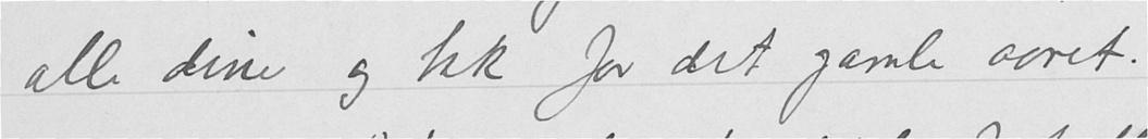alle dine og tak for det gamle aaret.
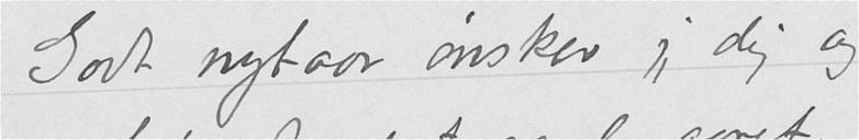Godt nytaar ønsker jeg dig og
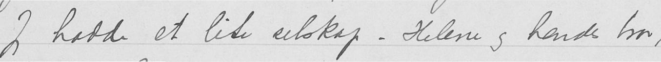Jeg hadde et lite selskap – Helene og hendes bror,
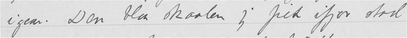igaar. Den blaa skaalen jeg fik ifjor stod
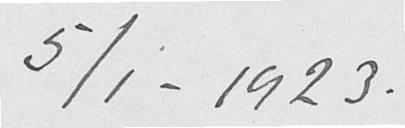5/1 - 1923.
